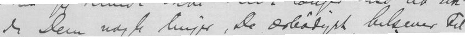de Dem nogle linjer, De ærbødigst hilsener til
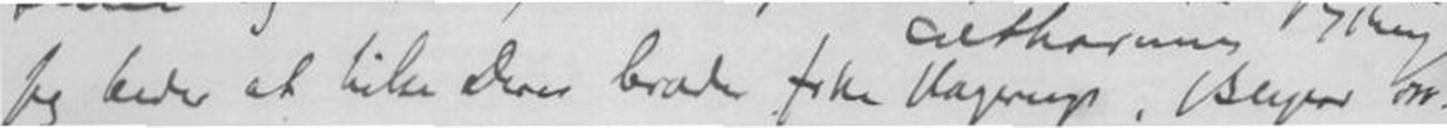Jeg beder at hilse Deres broder frk. Hagerup, Beyer osv.
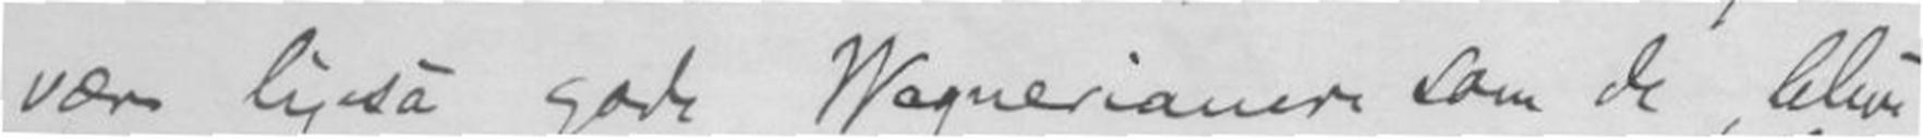være ligeså gode Wagnerianere som de, blive
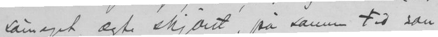såmeget ægte skjønt, på samme tid som
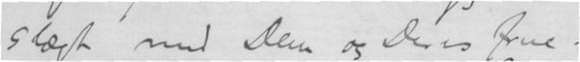slægt med Dem og Deres frue.
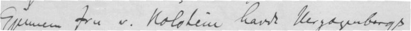Gjennem fru v. Kolstein havde Herzogenbergs
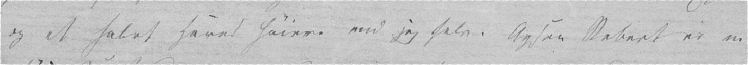og et halvt Hoved høiere end jeg selv. Ogsaa Robert er en
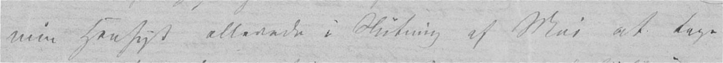min Hensigt allerede i Slutning af Mai at tage
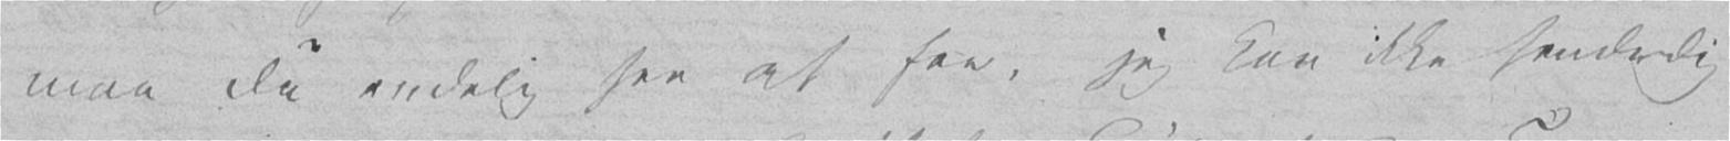maa Du endelig see at faa, jeg kan ikke sende Dig
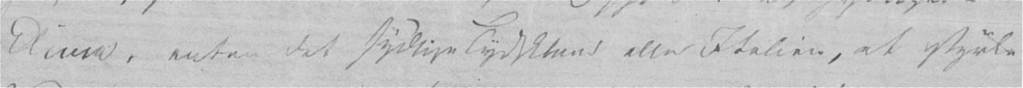Klima, enten det sydlige Tydskland eller Italien, at styrke
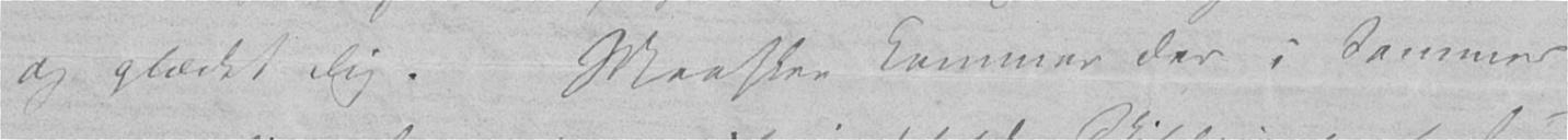og glædet Dig. Maaskee kommer der i Sommer
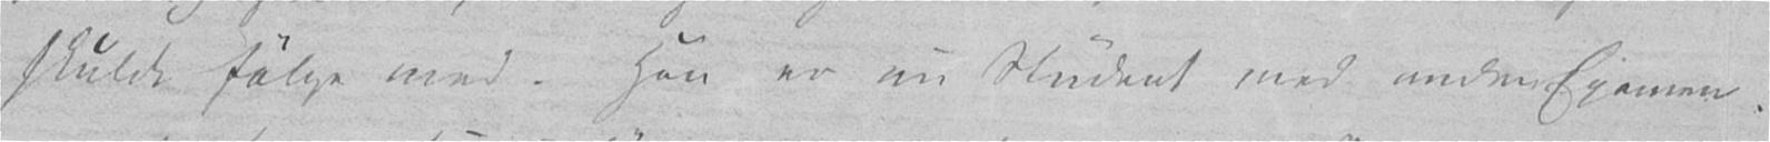skulde følge med. Han er nu Student med anden Examen
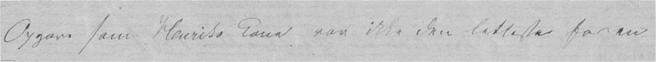Opgave som Henriks Kone var ikke den letteste for en
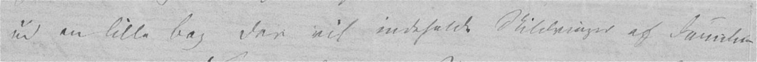ud en lille Bog der vil indeholde Skildringer af Familien
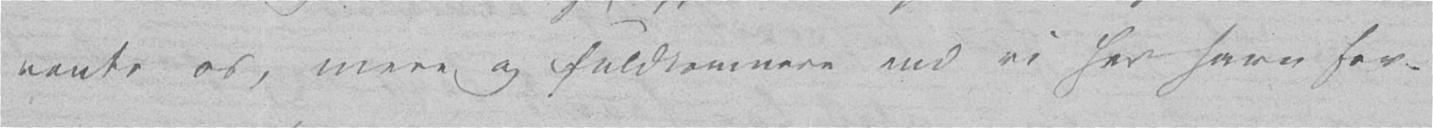vente os, mere og fuldkomnere end vi her have for-
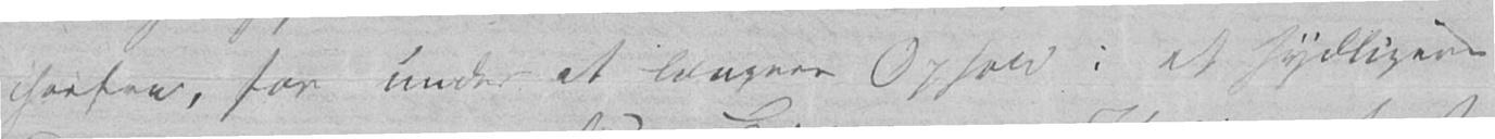herfra, for under et længere Ophold i et sydligere
